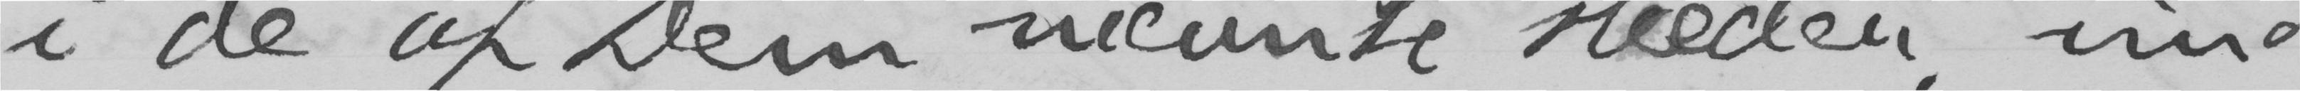i de af Dem nævnte stæder, und-
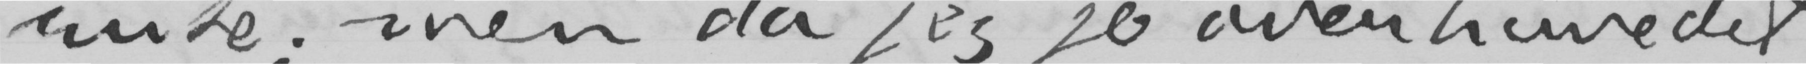reise, men da jeg jo overhovedet
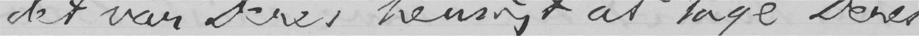det var Deres hensigt at tage Deres
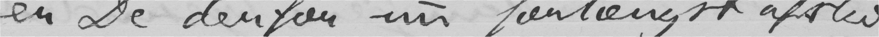er De derfor nu forlængst afsted.
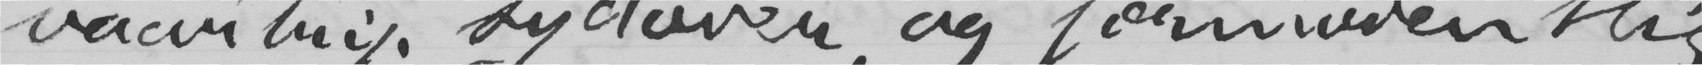vaartrip sydover, og formodentlig
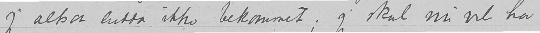jeg altsaa endda ikke bekommet; jeg skal nu vel
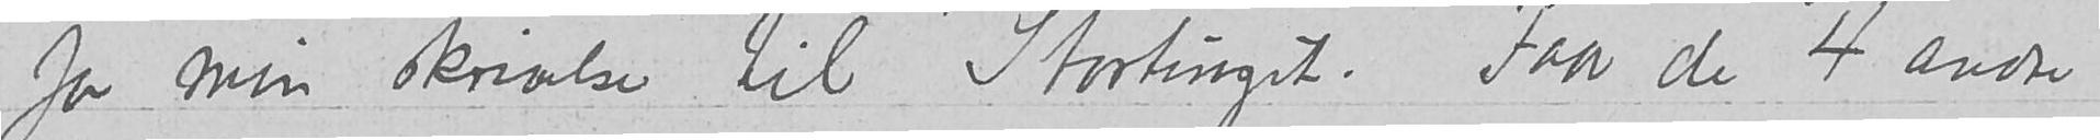for min skrivelse til Stortinget.  Faar de 4 andre
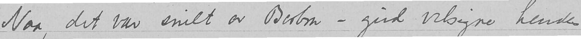Naa, det var snilt av Barbra - gud velsigne hendes
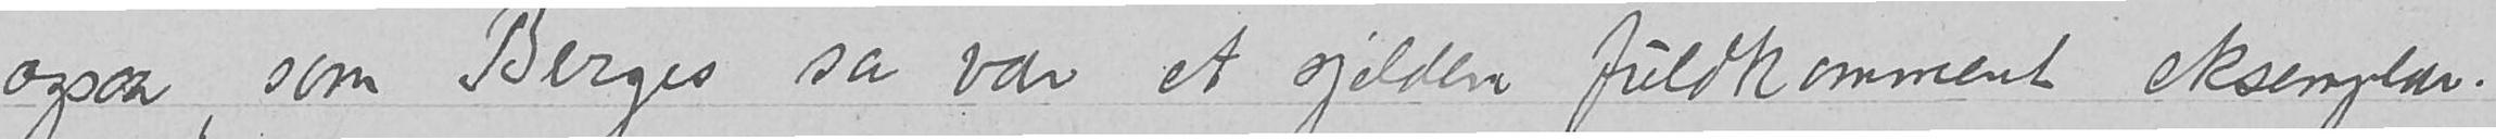ogsaa, som Berges sa var et sjelden fuldkomment eksemplar.
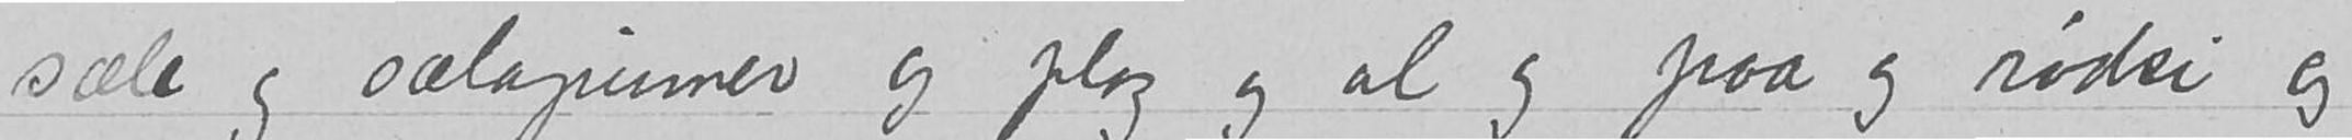sæle og sælepinner og plog og al og paa og rødsi og
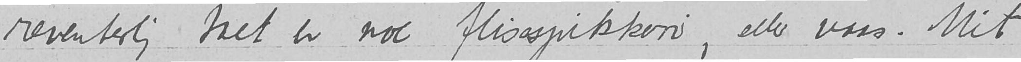reventerlig talt er noe flisespikkeri, eller vaas. Mit
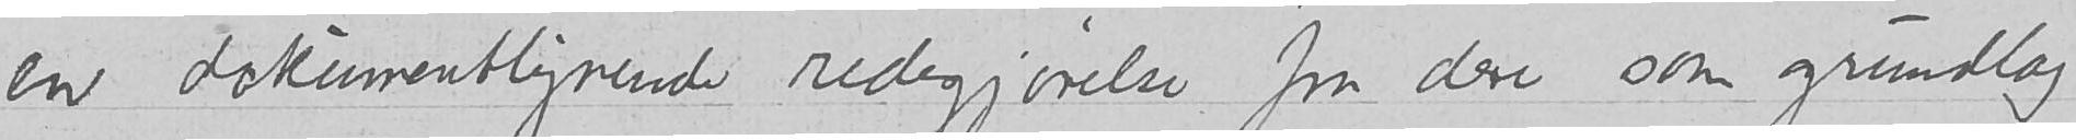ha en dokumentlignende redegjørelse fra dere som grundlag
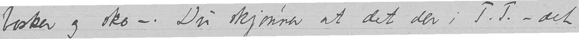tasker og sko -. Du skjønner at det der i T.T. -
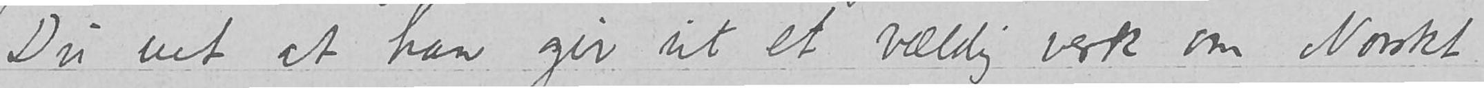Du vet at han gir ut et vældig verk om Norskt
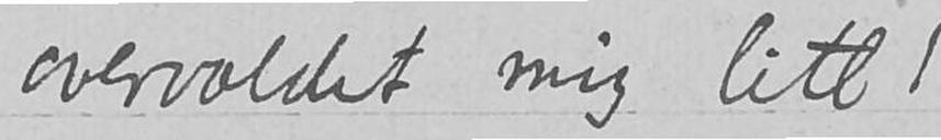det overvældet mig litt!
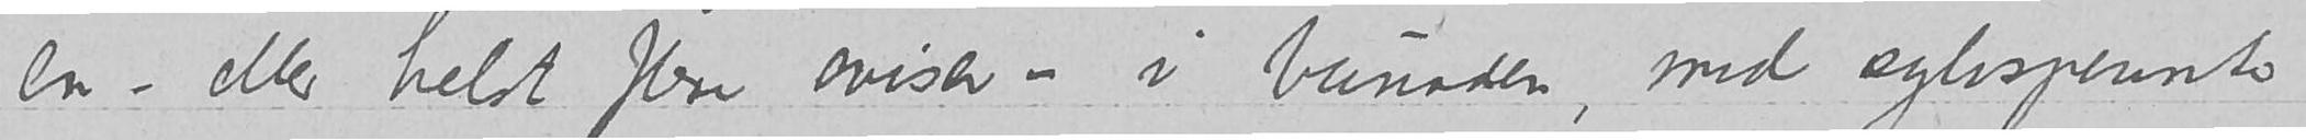en – eller helst flere aviser -i bunaden, med sylvspennte
